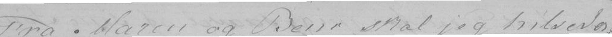Fra Maren og Bene skal jeg hilse Jer,
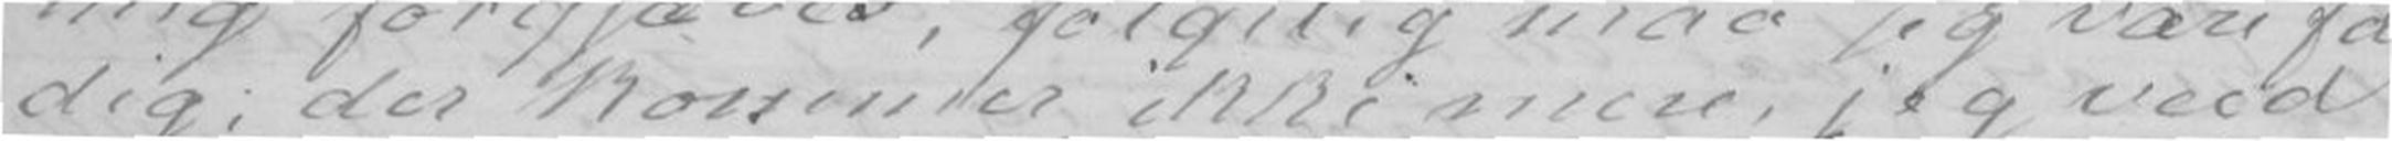dig; der kommer ikke mere, jeg veed
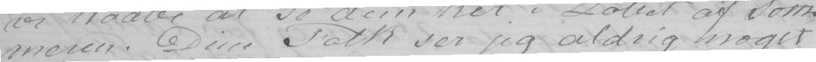meren. Dine Folk ser jeg aldrig noget
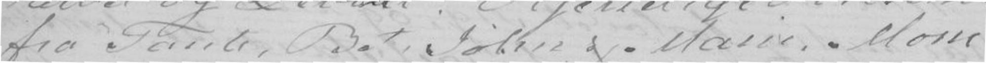fra Tante, Bet, John og Marie, Mone
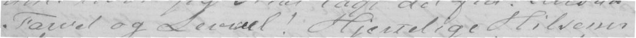Farvel og Levvel! Hjertelige Hilsener
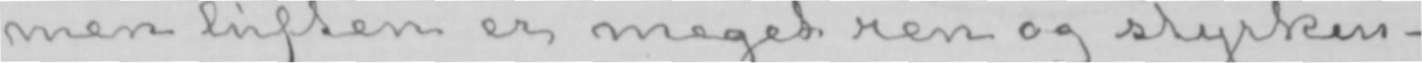men luften er meget ren og styrken-
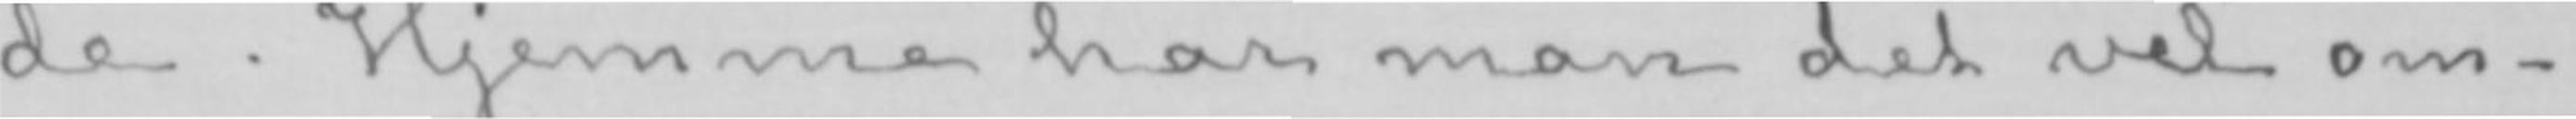de. Hjemme har man det vel om-
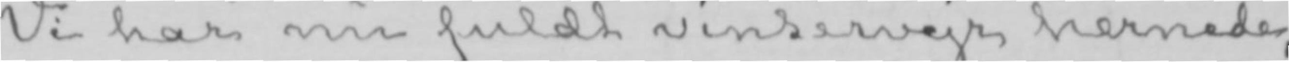Vi har nu fuldt vintervejr hernede,
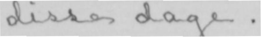disse dage.
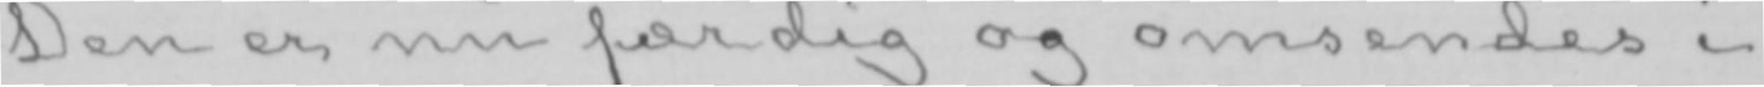Den er nu færdig og omsendes i
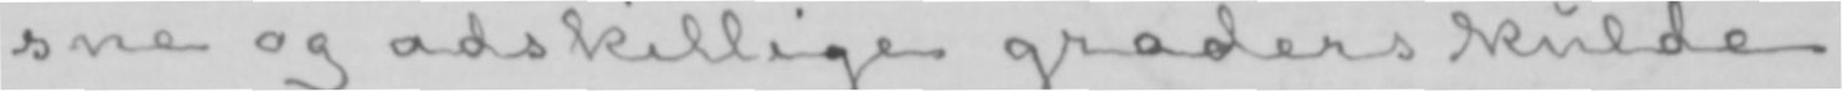sne og adskillige graders kulde;
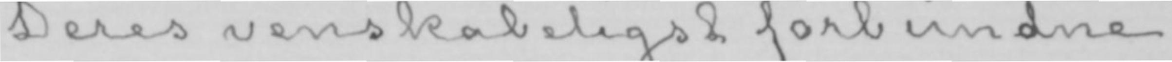Deres venskabeligst forbundne
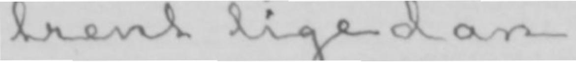trent ligedan.
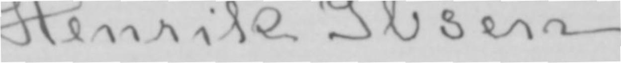Henrik Ibsen.
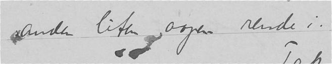anden liten aapen rende i.
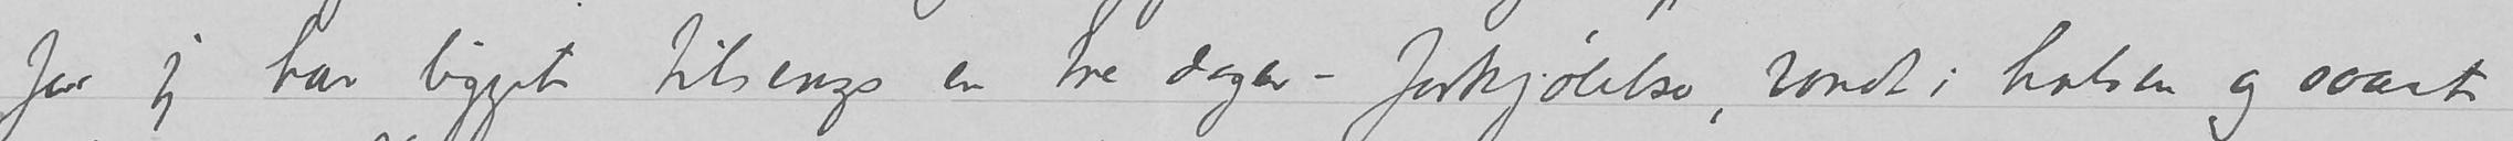for jeg har ligget til sengs en tre dager – forkjølelse, vondt i halsen og saart
